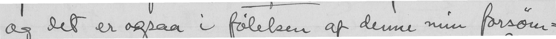og det er ogsaa i følelsen af denne min forsøm-
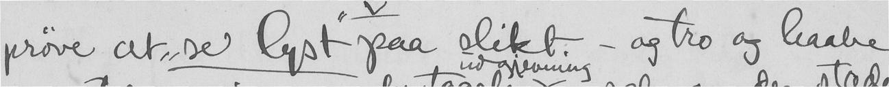prøve at "se lyst" paa slikt - og tro og haabe
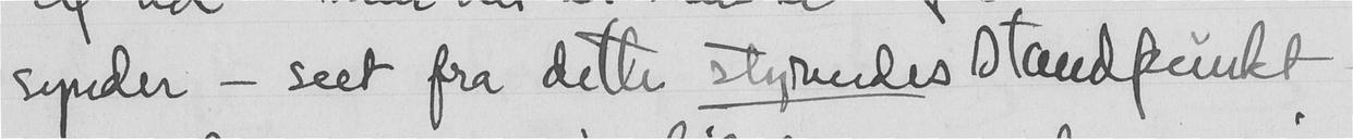synder - seet fra dette styrendes standpunkt
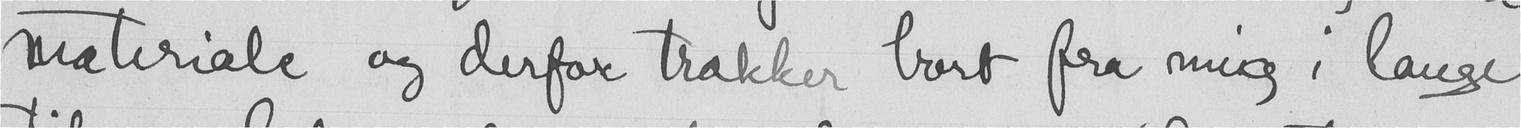materiale og derfor trækker bort fra mig i lange
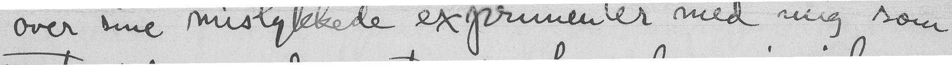over sine mislykkede exprimenter med mig som
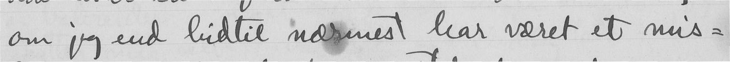om jeg end hidtil nærmest har været et mis-
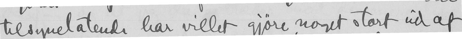tilsynelatende har villet gjøre noget stort ud af
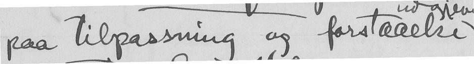paa tilpassning og forstaaelse,
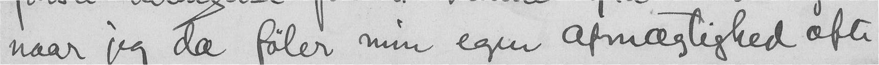naar jeg da føler min egen afmægtighed ofte
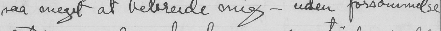saa meget at bebreide mig - uden forsømmelse
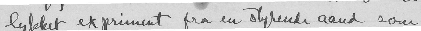lykket expriment fra en styrende aand som
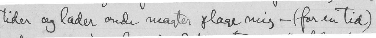tider og lader onde magter plage mig, - (for en tid)
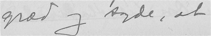græd og sagde, at
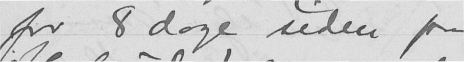for 8 dage siden paa
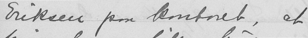Eriksen paa kontoret, at
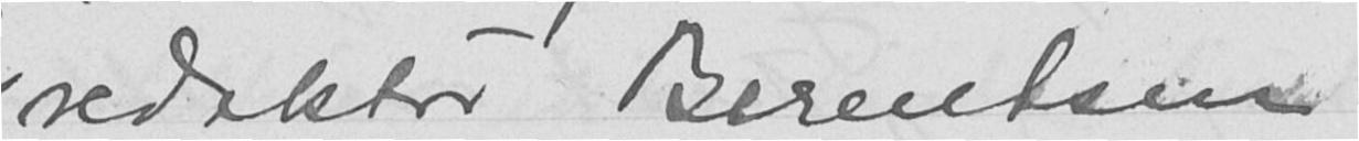redaktør Berentsen
